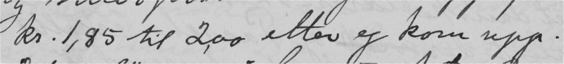kr. 1,85 til 2,00 etter eg kom upp.
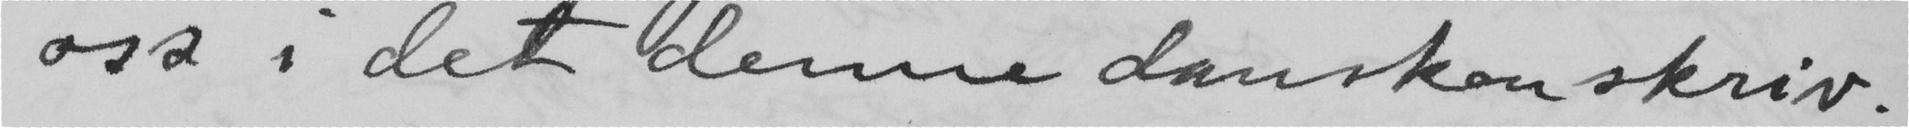oss i det denne dansken skriv.
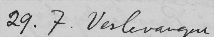29.7. Veslevangen
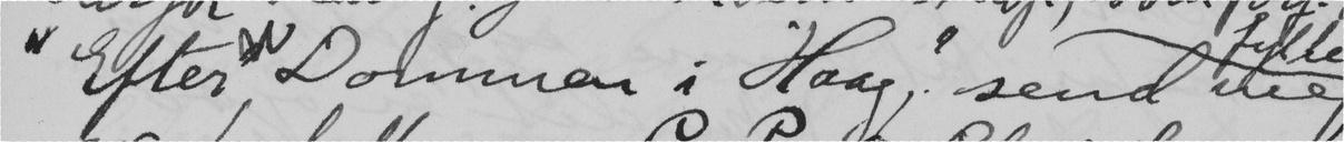"Efter Dommen i Haag", send meg
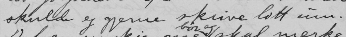skulde eg gjerne skrive litt um.
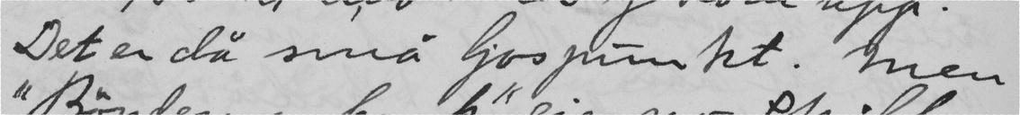Det er då små ljospunkt. Men
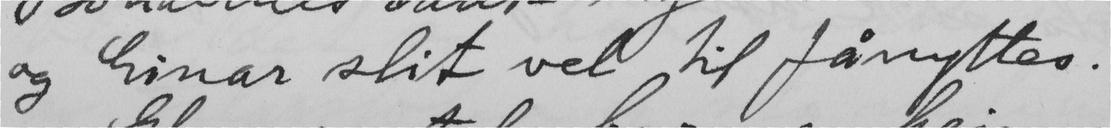og Einar slit vel til fånyttes.
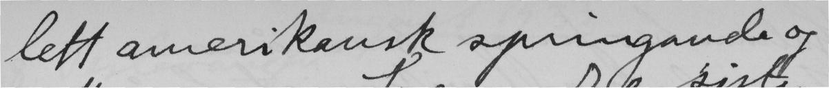lett amerikansk springande og
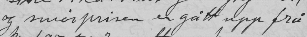og smörprisen er gått upp frå
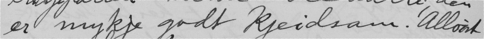er mykje godt kjeidsam. Alloit
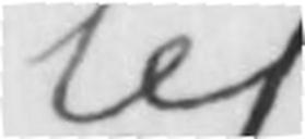lel
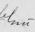nu
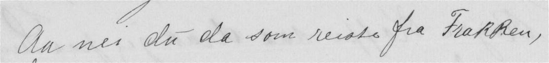Aa nei du da som reiste fra Frakken,
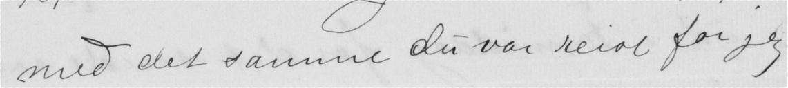med det samme du var reist for jeg
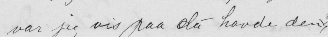var jeg vis paa da - havde den, nu
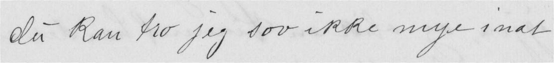du kan tro jeg sov ikke mye inat
